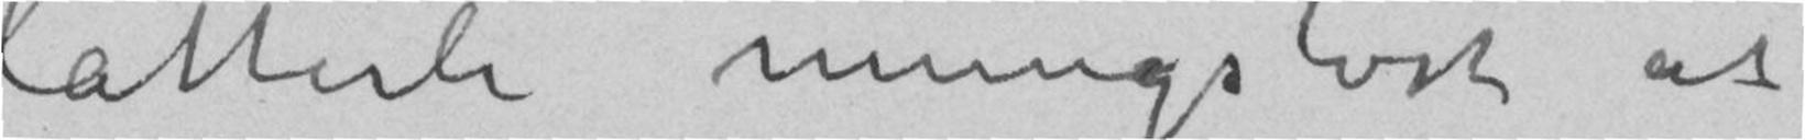latterli meningsløst at
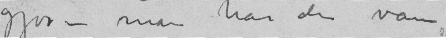gjør – man har da vaner
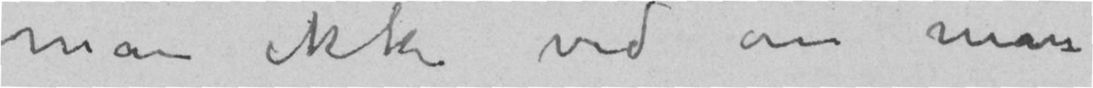man ikke ved om man
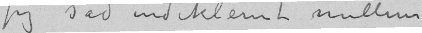jeg sad indeklemt mellem
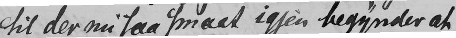til der nu saa smaat igjen begynder at
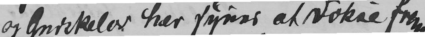og Gudskelov her synes at vokse frem
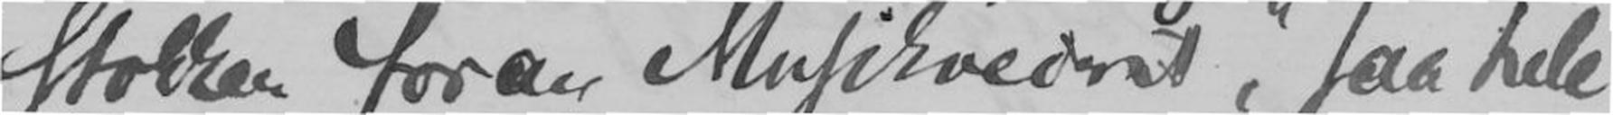stokken foran Musikvedret, "saa hele
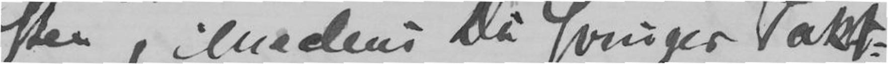stet, medens Du svinger Takt-
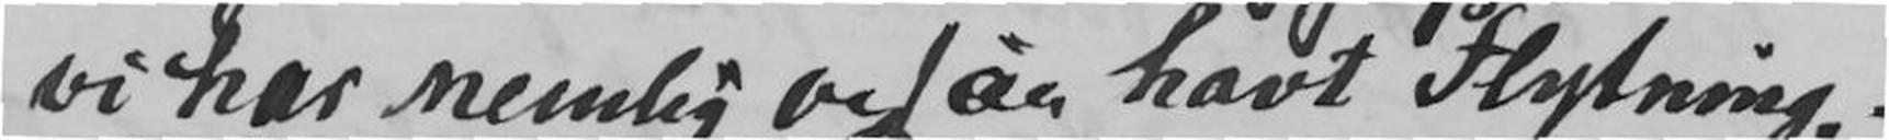vi har nemlig ogsaa havt Flytning, -
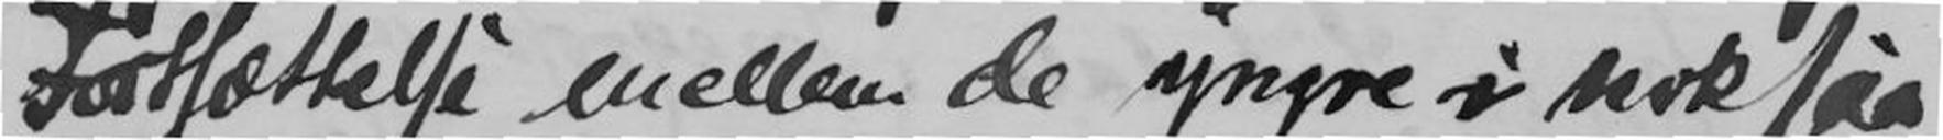Fortsættelse mellem de yngre i nok saa
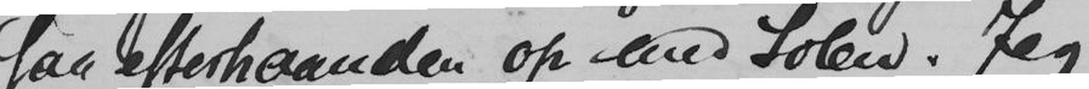saa efterhaanden op med Solen. Jeg
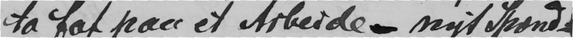ta fat paa et Arbeide - nyt Spænd-
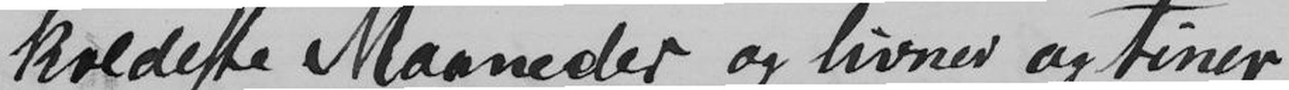koldeste Maaneder og livner og tiner
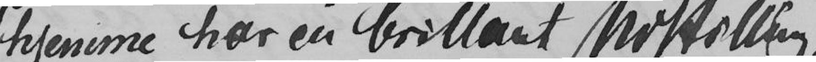hjemme har en brillant Udstilling,
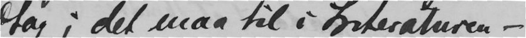tag; det maa til i Literaturen -
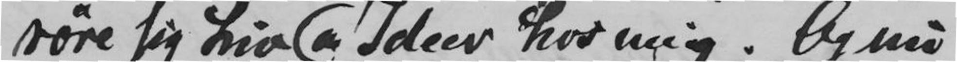røre sig Liv og Ideer hos mig. Og nu
